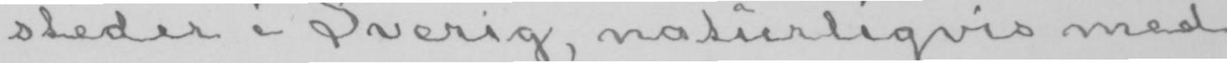steder i Sverig, naturligvis med
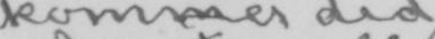kommer did
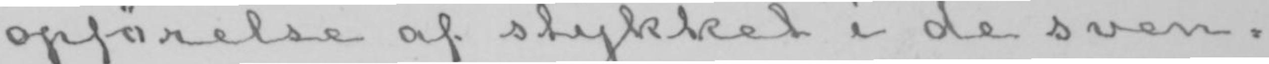opførelse af stykket i de sven-
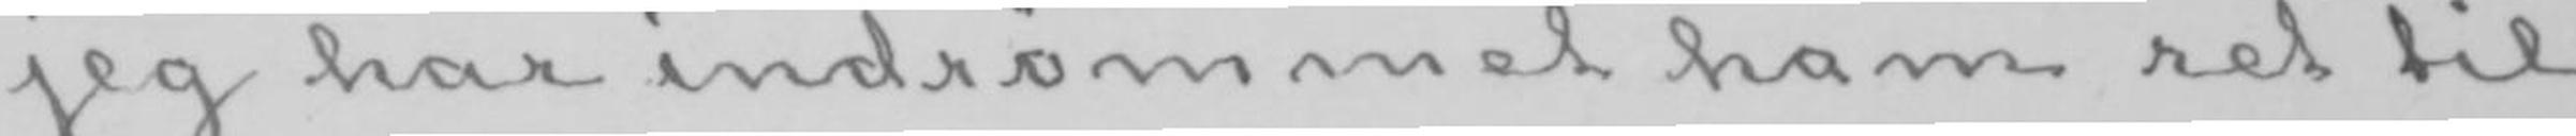jeg har indrømmet ham ret til
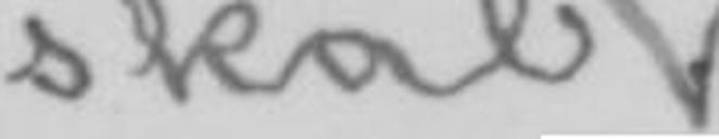skab
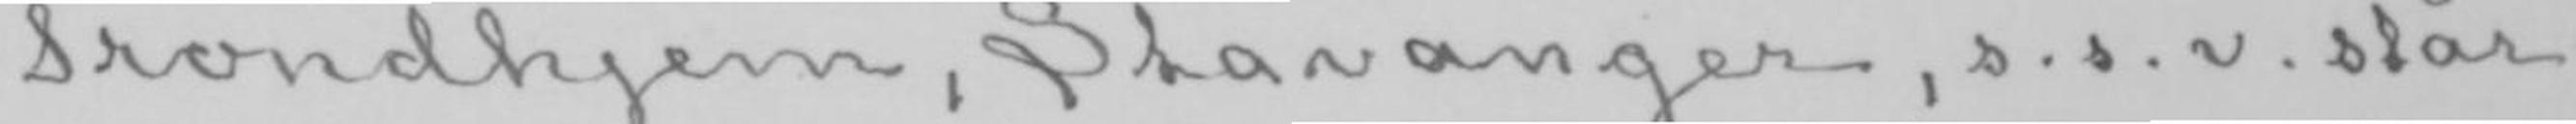Trondhjem, Stavanger, s. s. v. står
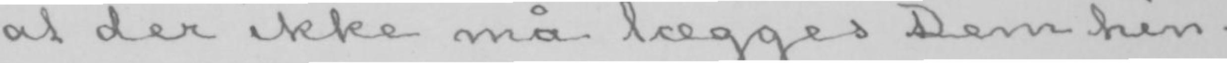at der ikke må lægges Dem hin-
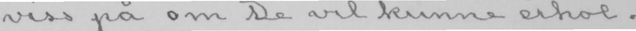viss på om De vil kunne erhol-
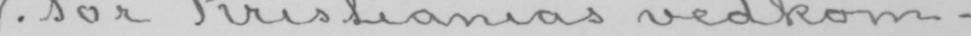. For Kristianias vedkom-
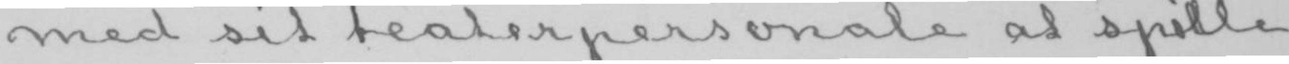med sit teaterpersonale at spille
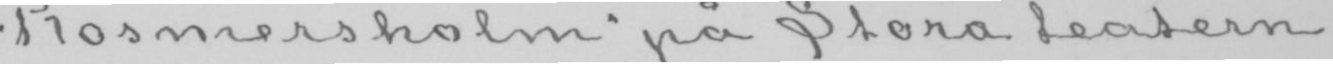«Rosmersholm» på Stora teatern
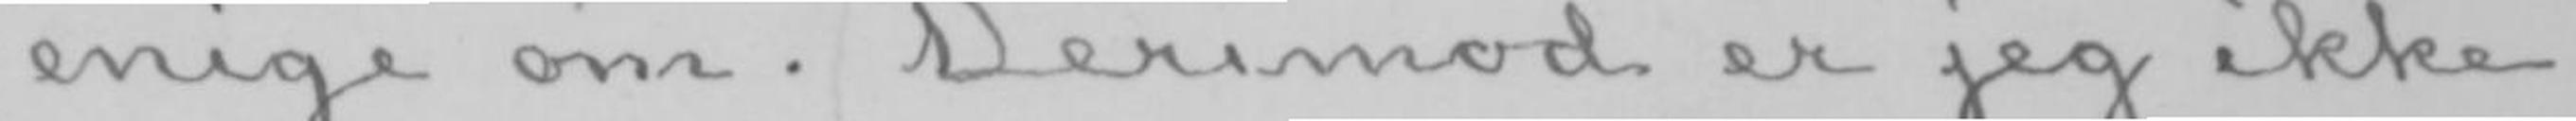enige om. Derimod er jeg ikke
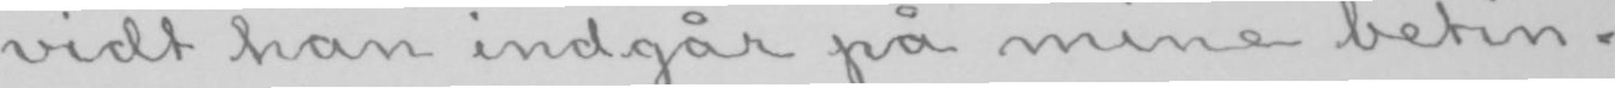vidt han indgår på mine betin-
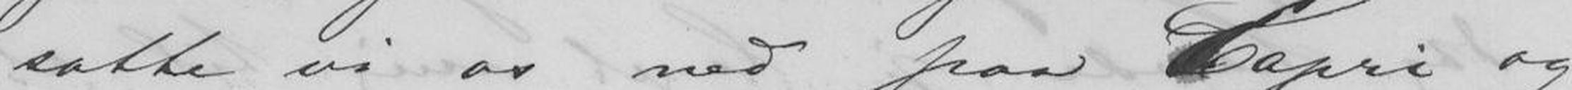satte vi os ned paa Capri og
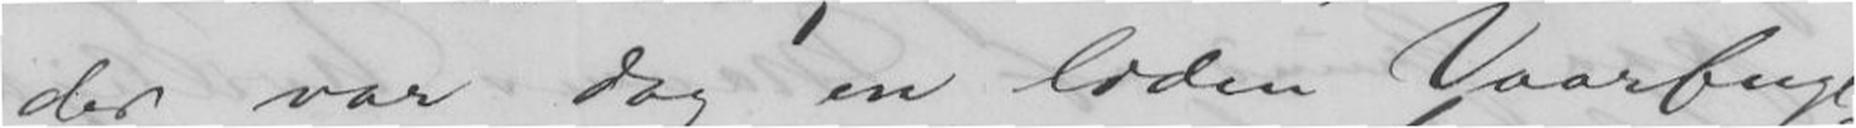der var dog en liden Vaarfugl i
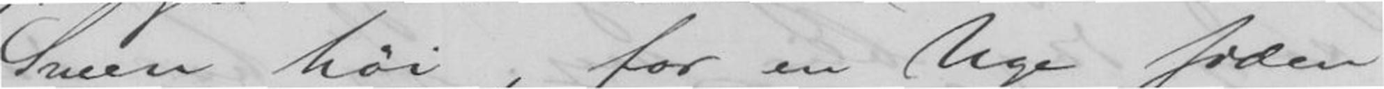Sneen høi, for en Uge siden
